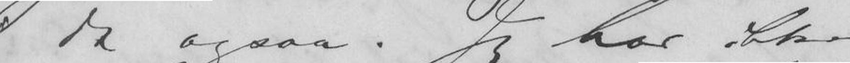jeg da ogsaa. Jeg har ikke
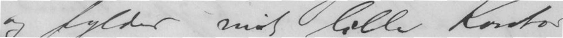og fylder mit lille Kontor
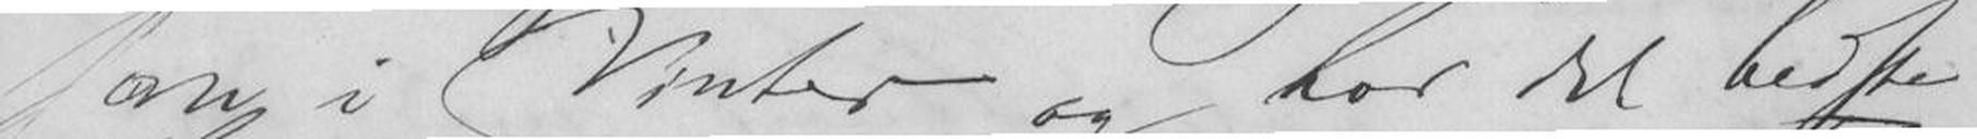som i Vinter og har det bedste
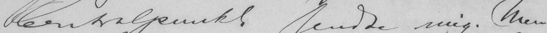Centralpunkt sendte mig. Men
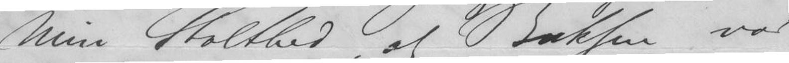min Stolthed, at Buksen var
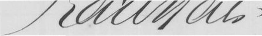Karl Hals
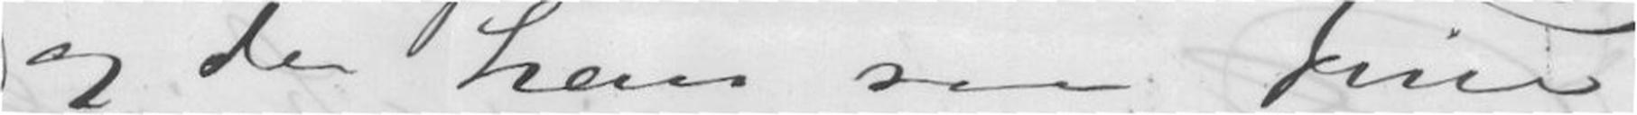og da han saa Dine
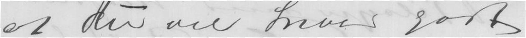at Du vil Leve godt
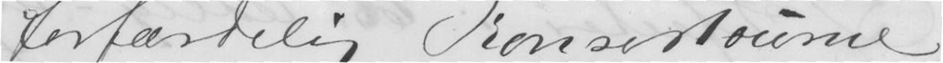forfærdelig Konsertourne
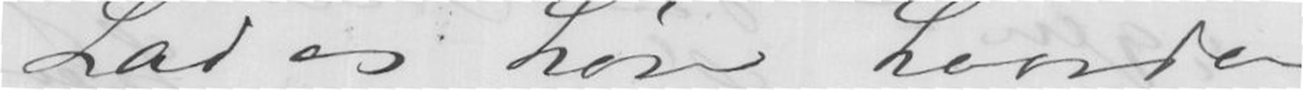Lad os høre hvordan
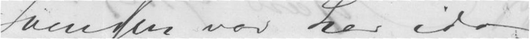Svendsen var her idag
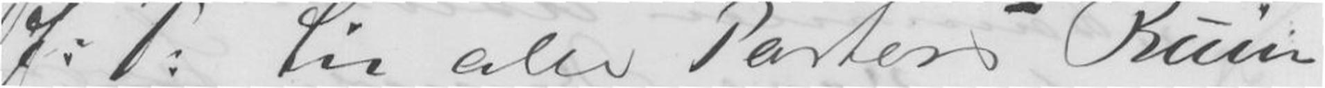f: T: til alle Parters Ruin.
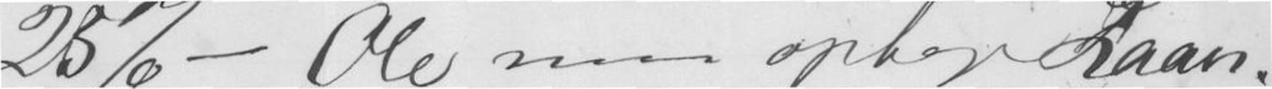25% - Ole maa optage Laan.
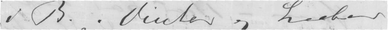i B. i Vinter og haaber
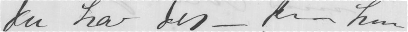du har det - Din hen
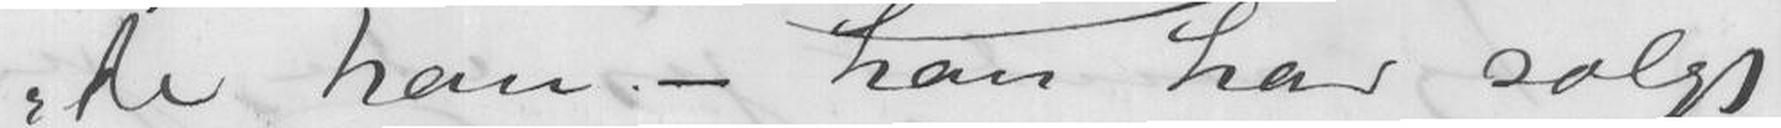de han. - han har solgt
